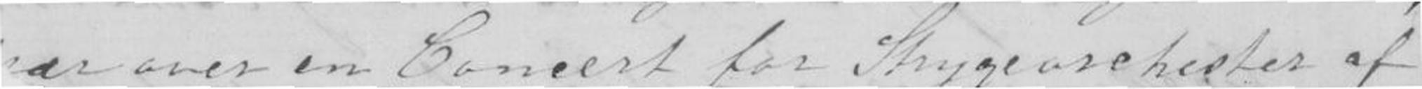ar over en Concert fot Strygeorchester af
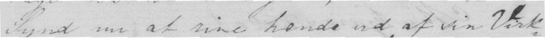Synd nu at rive hende ud af sin Virk-
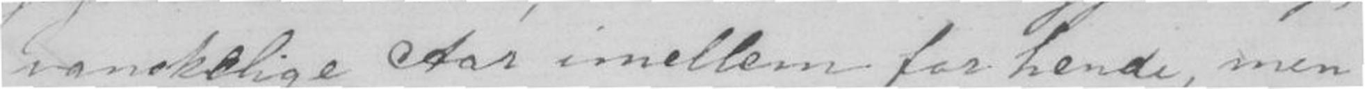vanskelige Aar imellem for hende, men
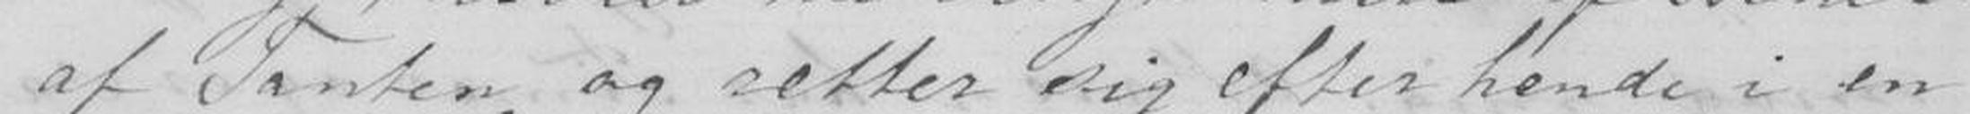af Tanten og sætter sig efter hende i en
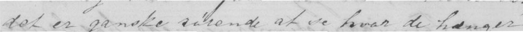det er ganske rørende at se hvad de hænger
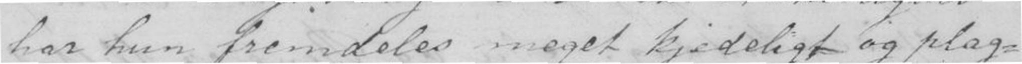har hun fremdeles meget kjedeligt og pag-
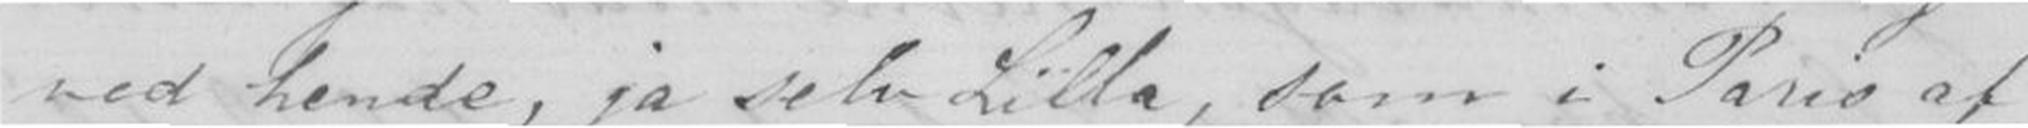ved hende, ja selv Lilla, som i Paris af
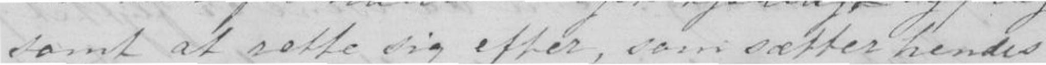somt at rette sig efter, som sætter hendes
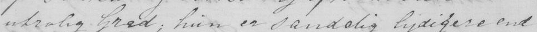utrolig Grad; hun er sandelig lydigere end
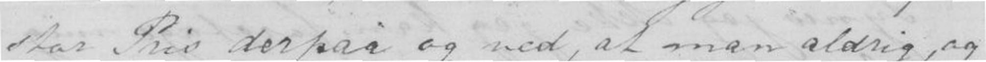stor Pris derpaa og ved, at man aldrig, og
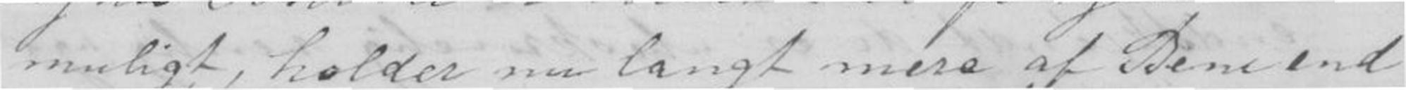muligt holder nu langt mere af Bene end
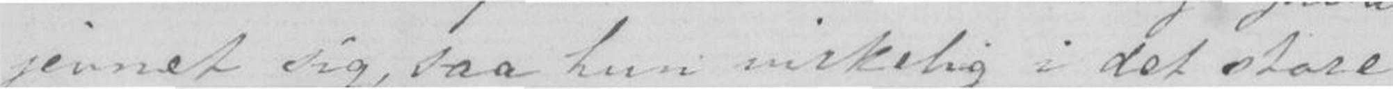jevnet sig, saa hun virkelig i det store
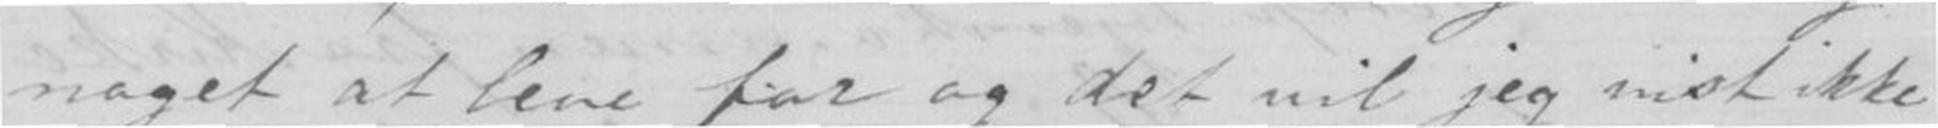noget at leve for og det vil jeg vist ikke
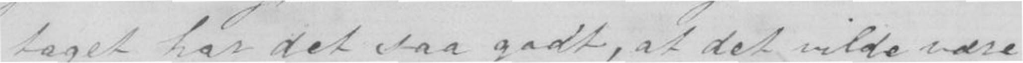taget har det saa godt, at det vilde være
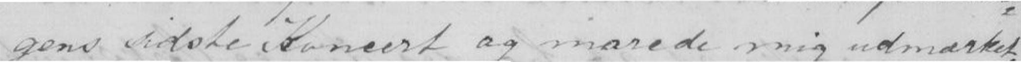gens sidste Koncert og morede mig udmærket
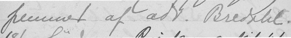fremmet af adv. Bredahl.
